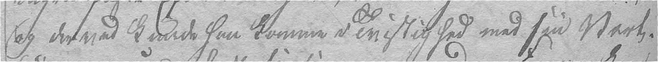og derved kunde han komme i Tvistighed med sin Vært.
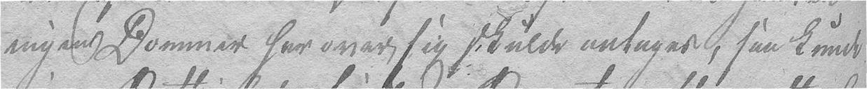ingen Dommer har over sig skulde antages, saa kunde
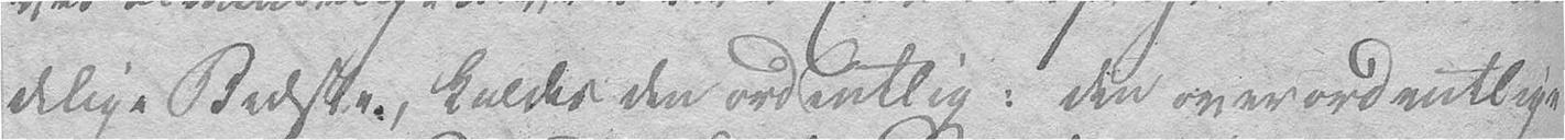delige Bedste, kaldes den ordentlig: den overordentlige
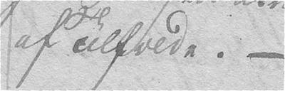af Tilfælde. –
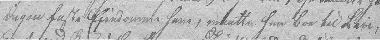ingen faste Eiendomme have, maatte han boe til Leie,
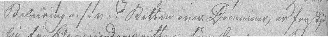Beleiring o:s:v:. Retten over Domainer er forskjel-
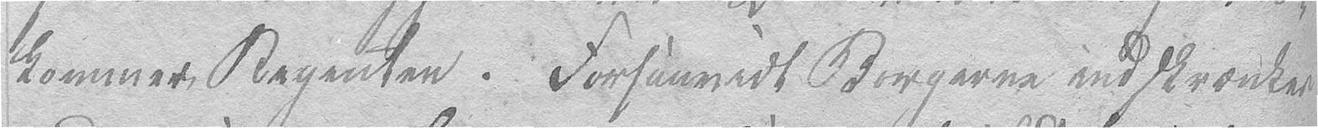kommer Regenten. Forsaavidt Borgerne indskrænkes
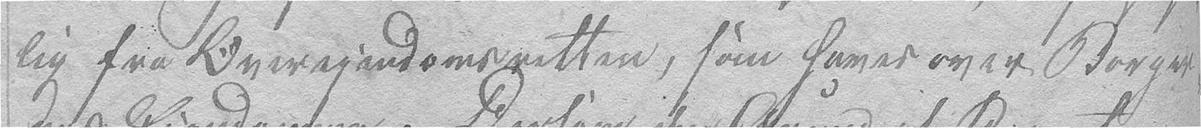lig fra Overeiendomsretten, som haves over Borger-
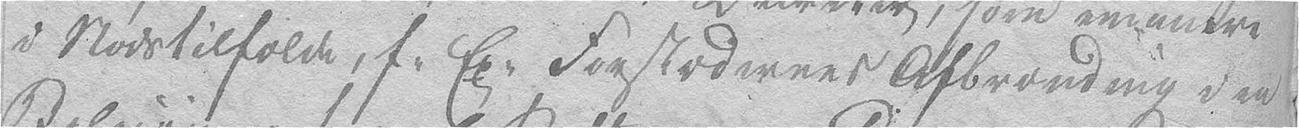i Nødstilfælde, f: Ex: Forstædernes Afbrænding i en
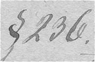§ 236.
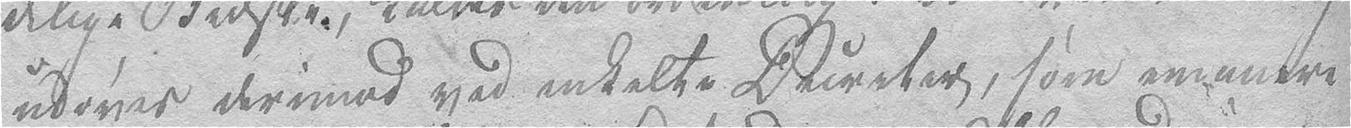udøves derimod ved enkelte Decreter, som anvendes
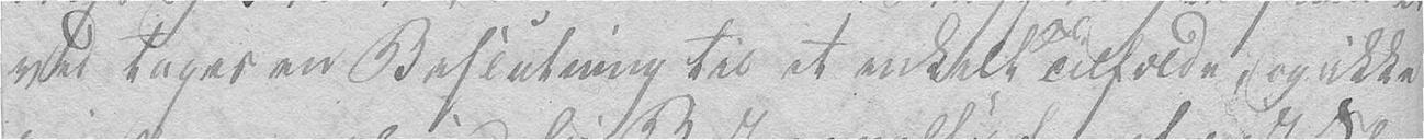ved tages en Beslutning til et enkelt Tilfælde, og ikke
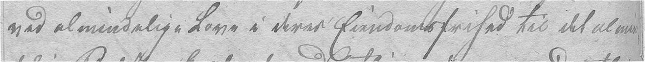ved almindelige Love i deres Eiendomsfrihed til det almin-
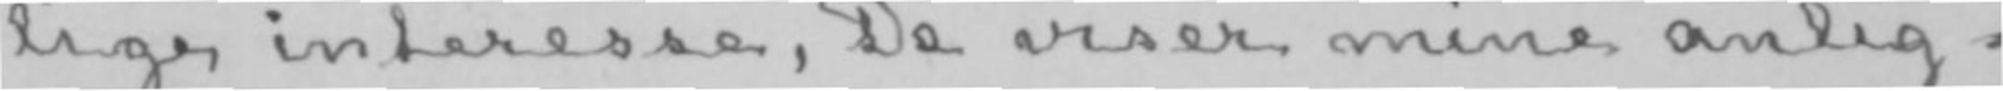lige interesse, De viser mine anlig-
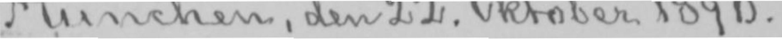München, den 22. Oktober 1890.
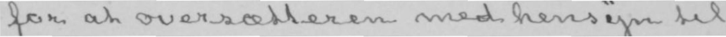for at oversætteren med hensyn til
